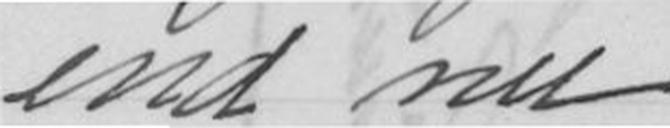end nu.
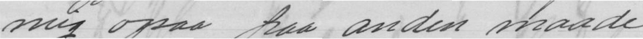mig ogsaa paa anden maade
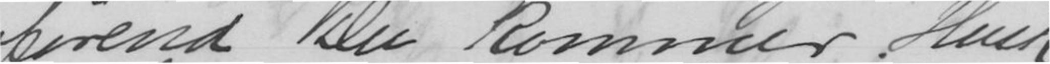førend Du kommer. Husk,
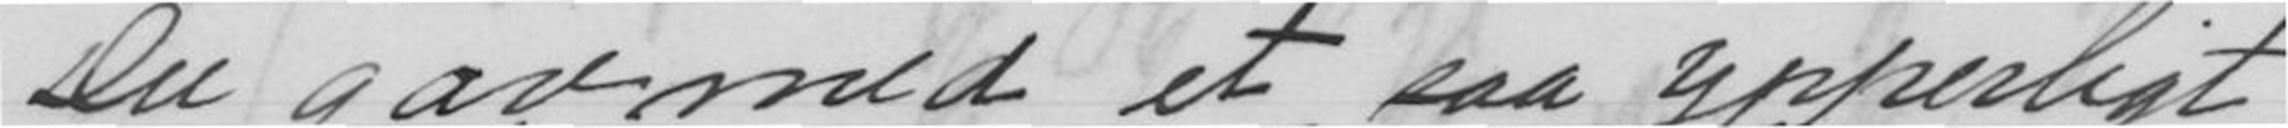Du gav med et saa ypperligt
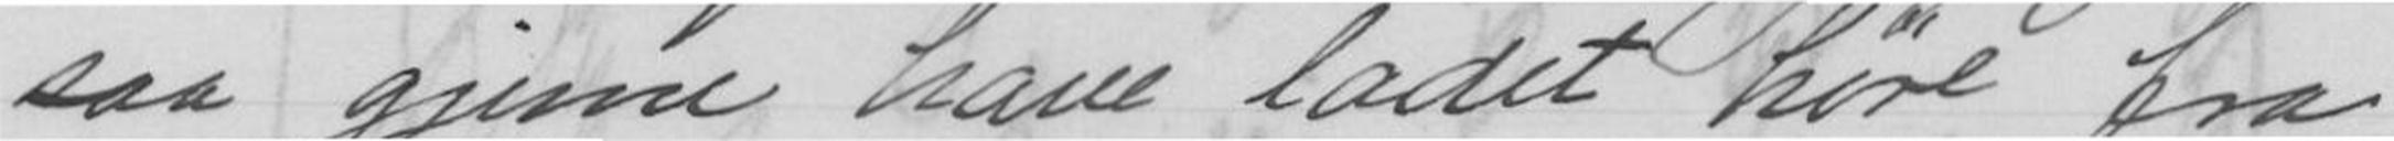saa gjerne have ladet høre fra
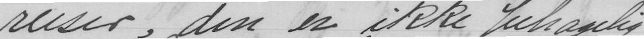reiser, den er ikke behagelig
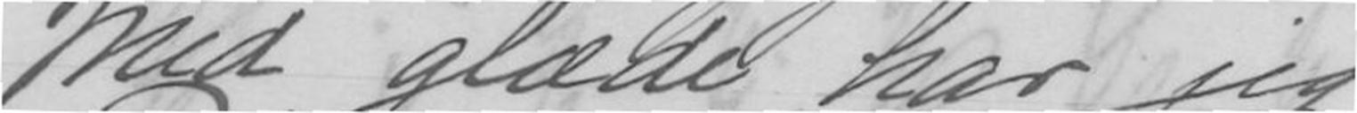Med glæde har jeg
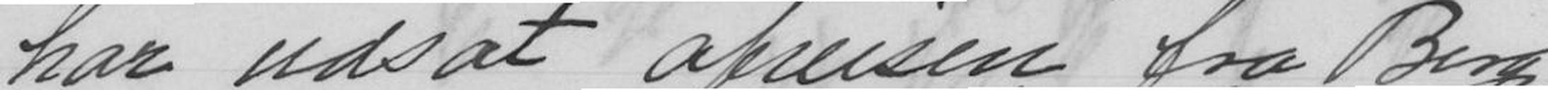har udsat afreisen fra Bergen
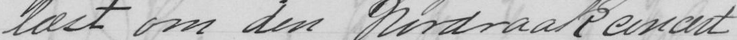læst om den Nordraakconcert
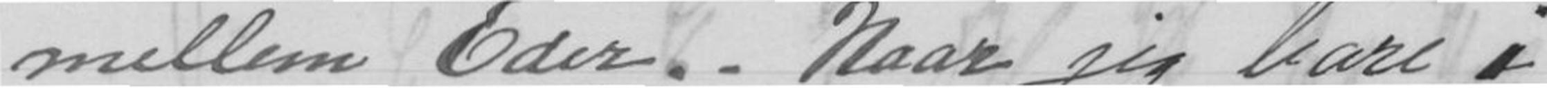mellem Eder. - Naar jeg bare i
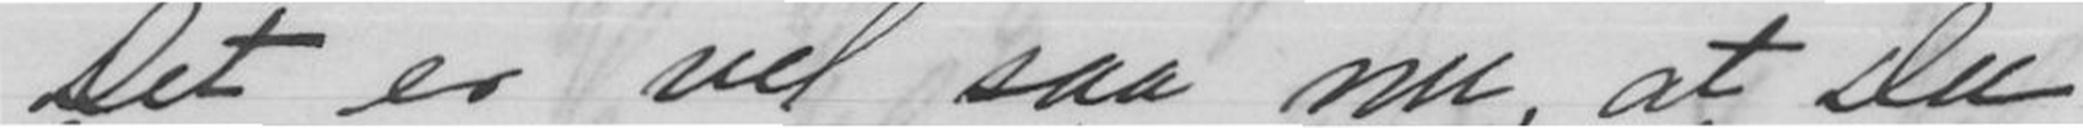Det er vel saa nu, at Du
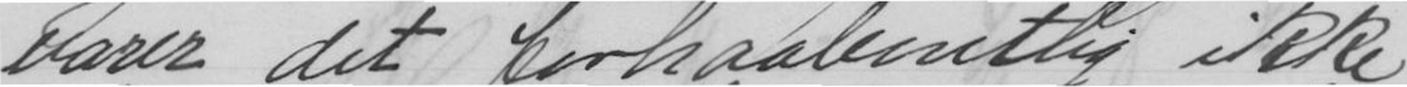varer det forhaabentlig ikke
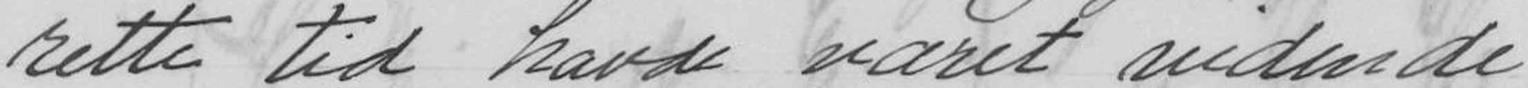rette tid havde været vidende
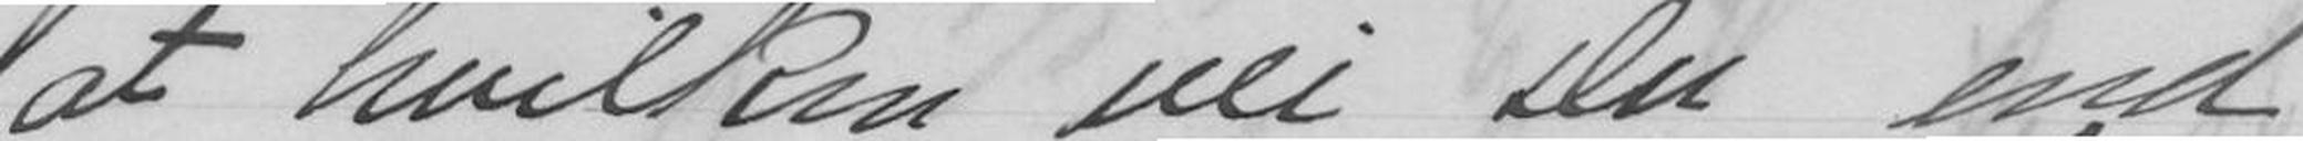at hvilken vei Du end
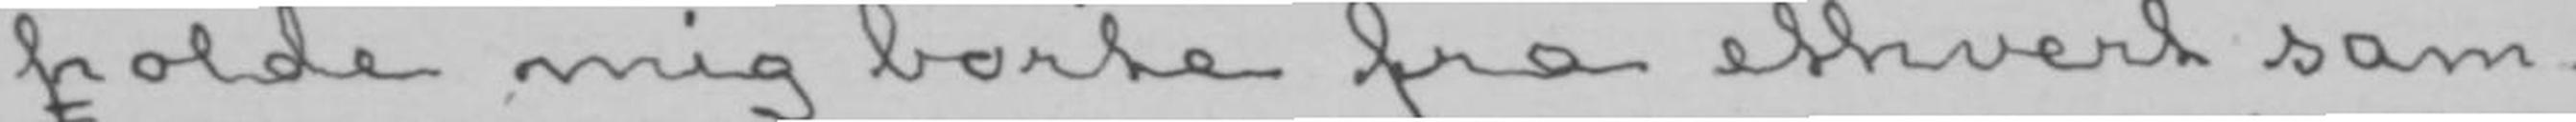fholde mig borte fra ethvert sam-
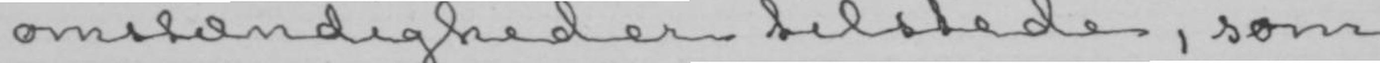omstændigheder tilstede, som
